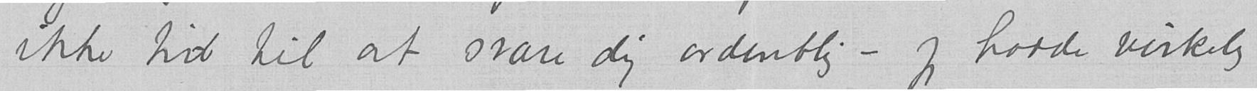ikke tid til at svare dig ordentlig - jeg havde virkelig
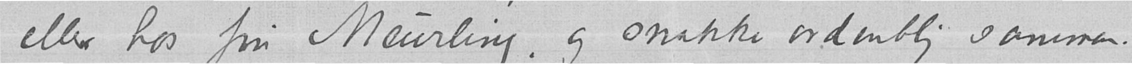eller hos fru Meurling, og snakke ordentlig sammen.
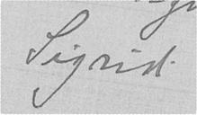Sigrid.
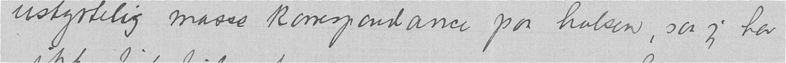ustyrtelig masse korrespondance paa halsen, saa jeg har
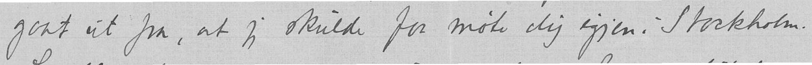gaat ut fra, at jeg skulde faa møte dig igjen i Stockholm.
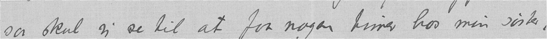saa skal vi se til at faa nogen timer hos min søster,
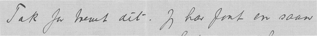Tak for brevet dit. Jeg har faat en saan
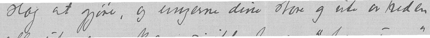slag at gjøre, og ungerne dine store og ute av reden
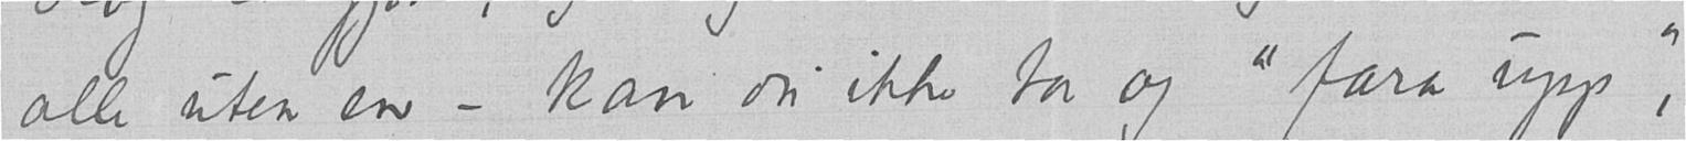alle uten en - kan du ikke ta og "fara upp",
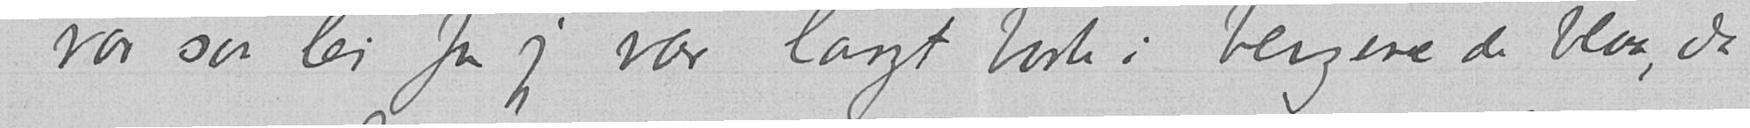Jeg var saa lei for jeg var langt borte i bergene de blaa, da
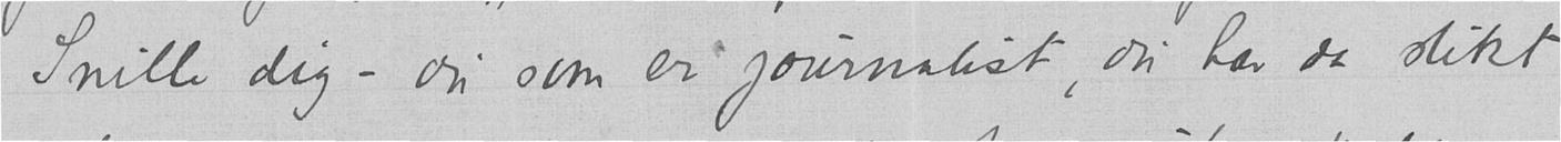Snille dig - du som er journalist, du har da slikt
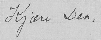Kjære Dea,
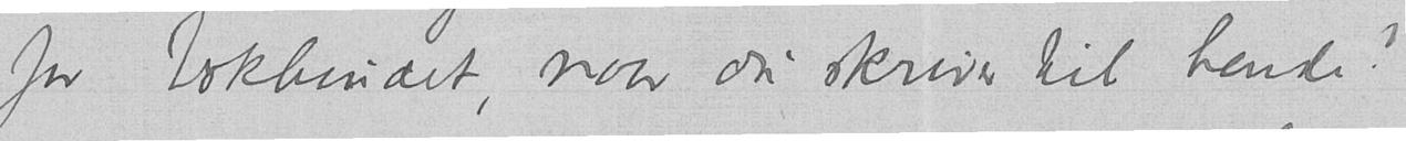for bokbindet, naar du skriver til hende!
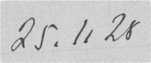25.11.28
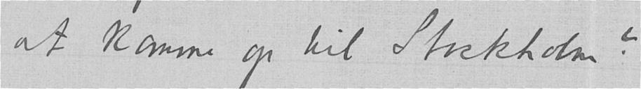at komme op til Stockholm?
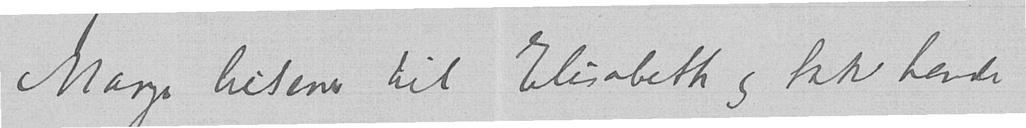Mange hilsener til Elisabeth og tak hende
